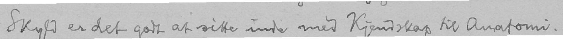Skyld er det godt at sitte inde med Kjendskap til Anatomi.
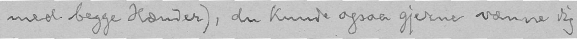med begge Hænder), du kunde ogsaa gjerne vænne dig
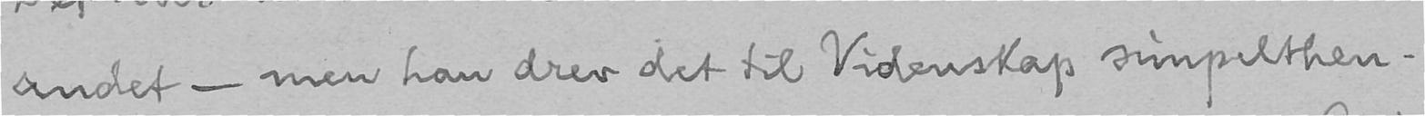andet - men han drev det til Videnskap simpelthen.
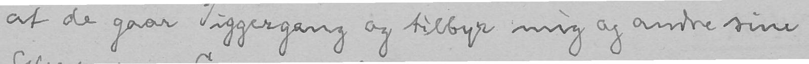at de gaar Tiggergang og tilbyr mig og andre sine
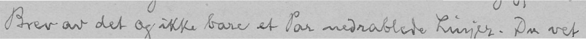Brev av det og ikke bare et Par nedrablede Linjer. Du vet
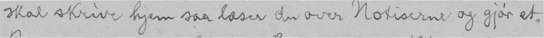skal skrive hjem saa læser du over Notiserne og gjør et
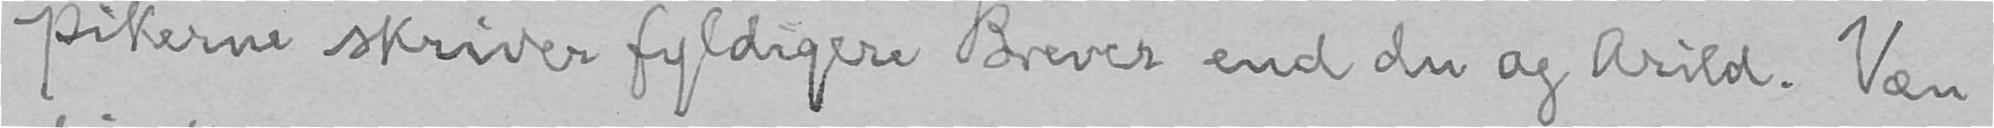pikerne skriver fyldigere Brever end du og Arild. Væn
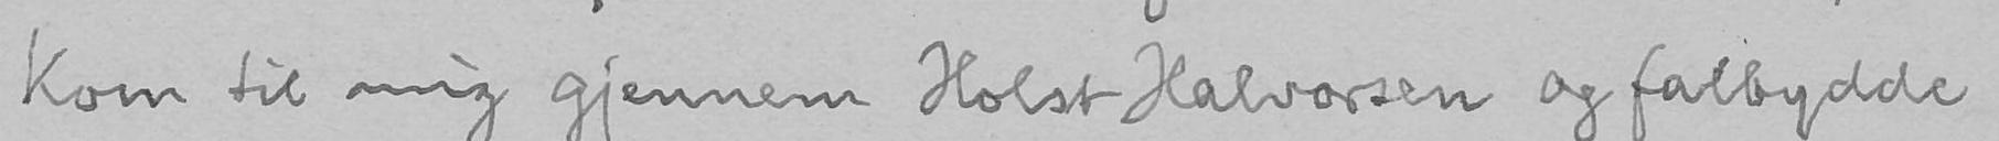kom til mig gjennem Holst Halvorsen og falbydde
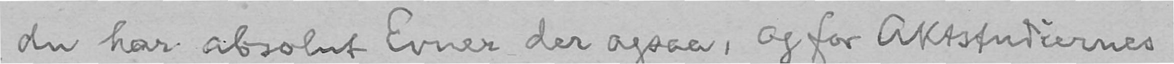du har absolut Evner der ogsaa, og for Aktstudiernes
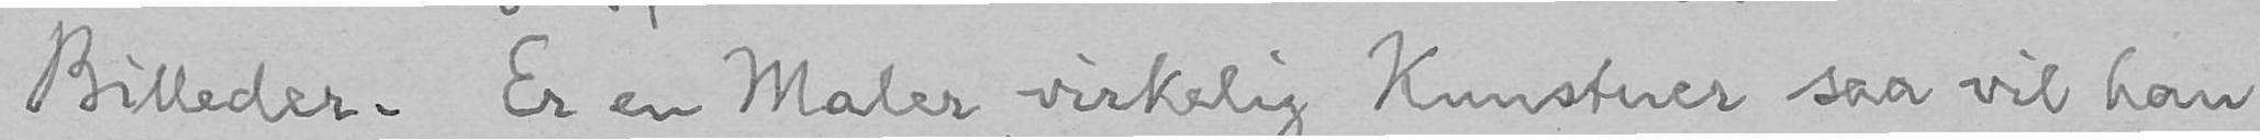Billeder. Er en Maler virkelig Kunstner saa vil han
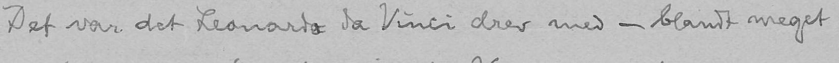Det var det Leonardo da Vinci drev med - blandt meget
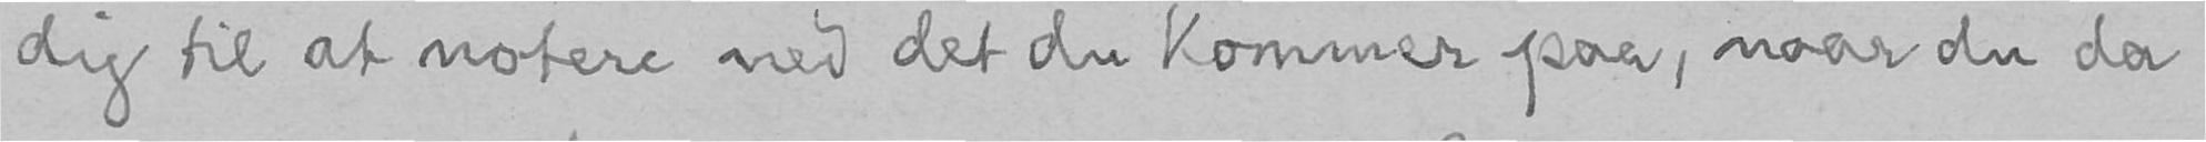dig til at notere ned det du kommer paa, naar du da
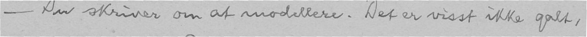- Du skriver om at modellere. Det er visst ikke galt,
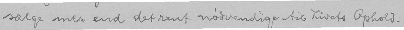sælge mer end det rent nødvendige til Livets Ophold.
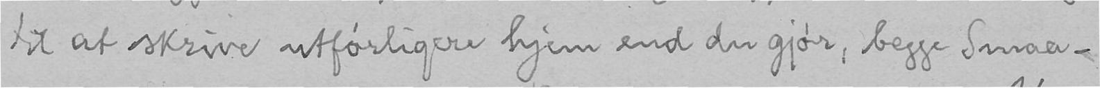til at skrive utførligere hjem end du gjør, begge Smaa-
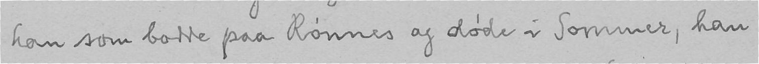han som bodde paa Rønnes og døde i Sommer, han
